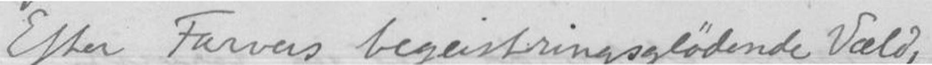Efter Farvers begeistringsglødende Væld,
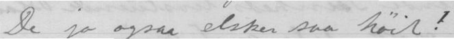De jo ogsaa elsker saa høit!
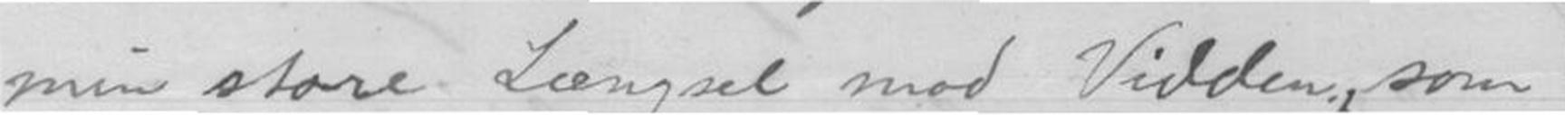min store Længsel mod Vidden, som
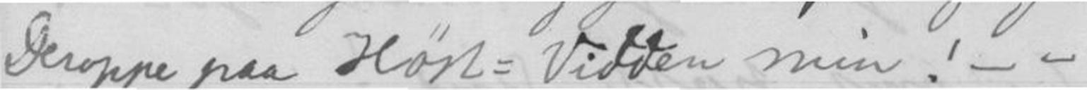Deroppe paa Høst-Vidden min! - -
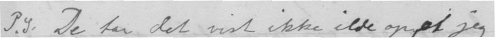P.S. De tar det vist ikke ilde op, at jeg
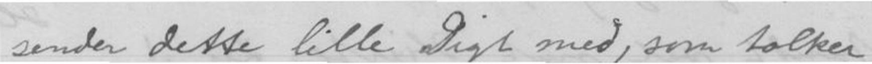sender dette lille Digt med, som tolker
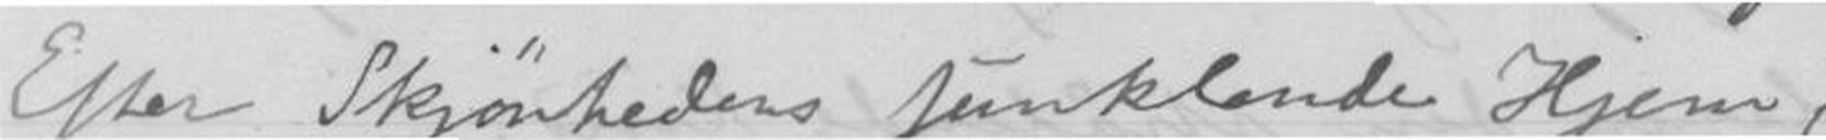Efter Skjøhedens funklende Hjem,
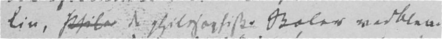Liv, Philo de philosophiske Skoler vedblev,
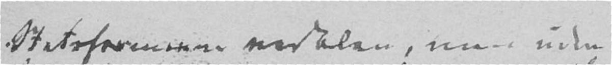Statsformerne vedblev, men uden
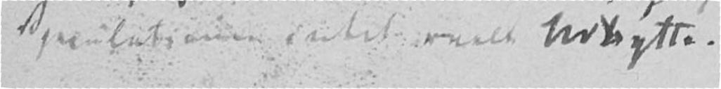Speculationen intet reelt Udbytte.
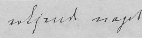erkjende noget
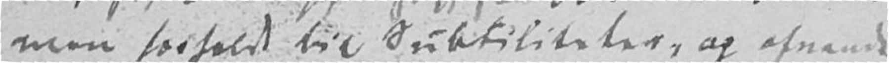men forfaldt til Subtiliteter, og afvandt
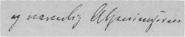og navnlig Athenienserne
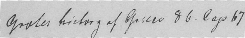Grotes History of Greece 8 B. Cap 67
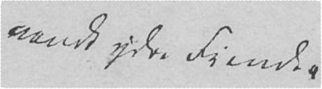vandt ydre Fiender
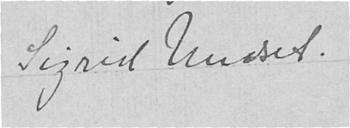Sigrid Undset.
